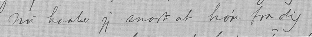Nu haaber jeg snart at høre fra dig
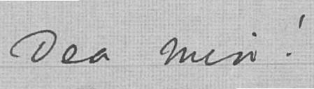Dea min!
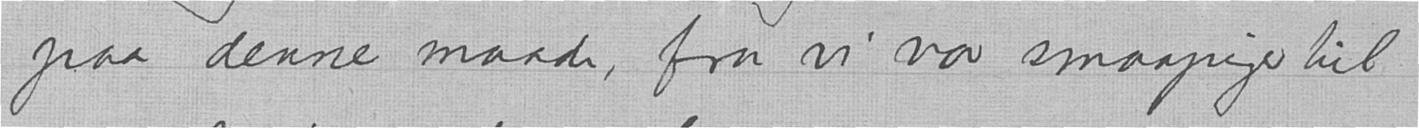paa denne maade, fra vi var smaapiger til
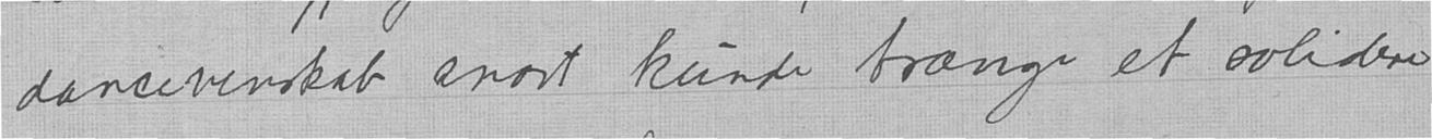dancevenskab snart kunde trænge et solidere
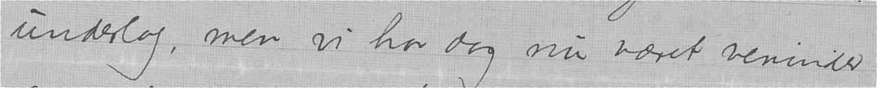underlag, men vi har dog nu været veninder
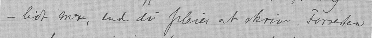— lidt mere, end du pleier at skrive. Forresten
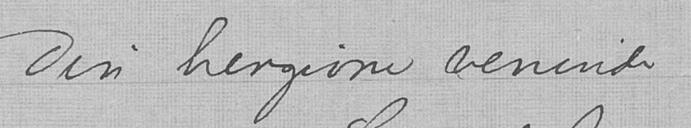Din hengivne veninde
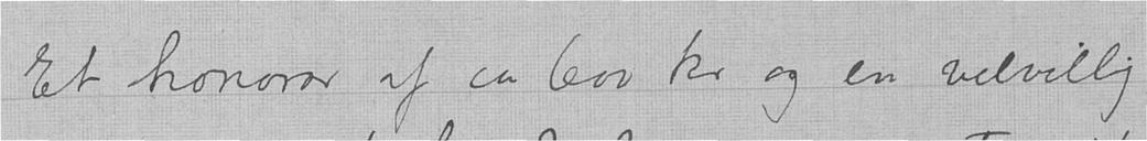Et honorar af ca. 600 kr og en velvillig
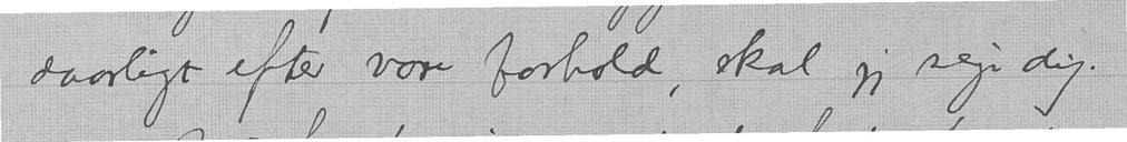daarligt efter vore forhold, skal jeg sige dig.
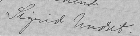Sigrid Undset.
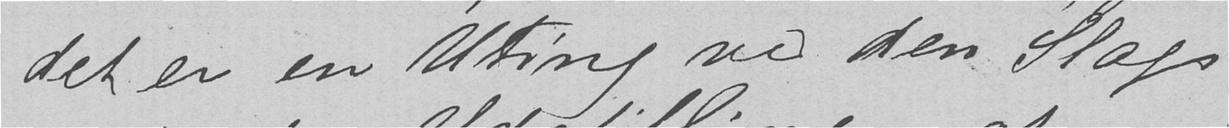det er en Uting ved den Slags
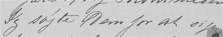Jeg søgte Dem for at sige,
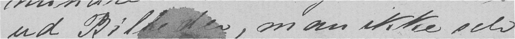ud Billeder, man ikke selv
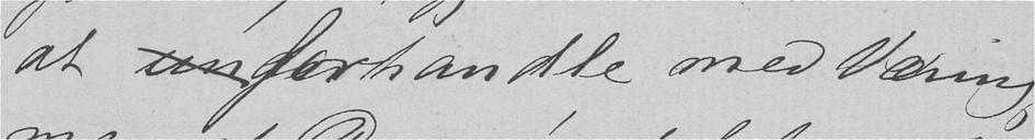at unforhandle med Væring,
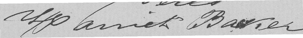Harriet Backer
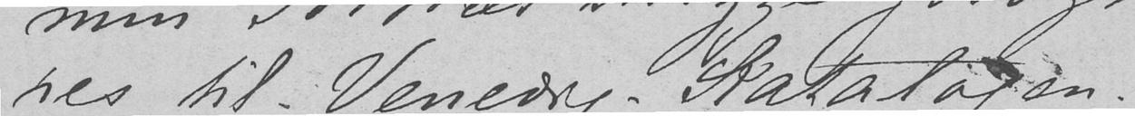res til Venedig-Katalogen.
